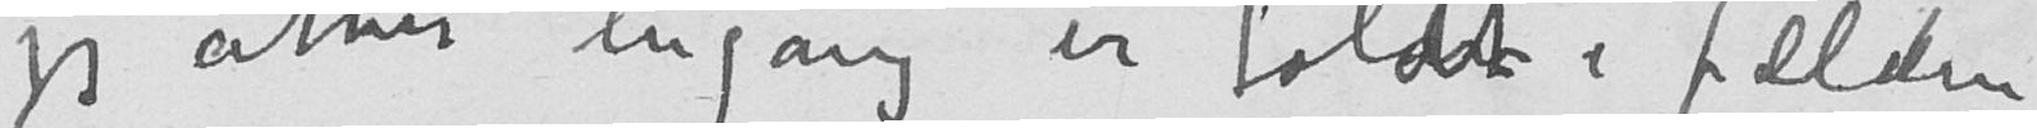jeg atter engang er faldt i fælden
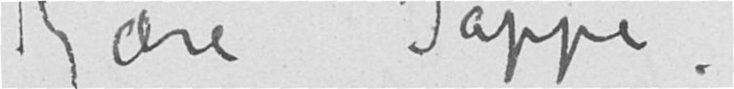Kjære Jappe!
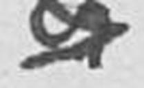er
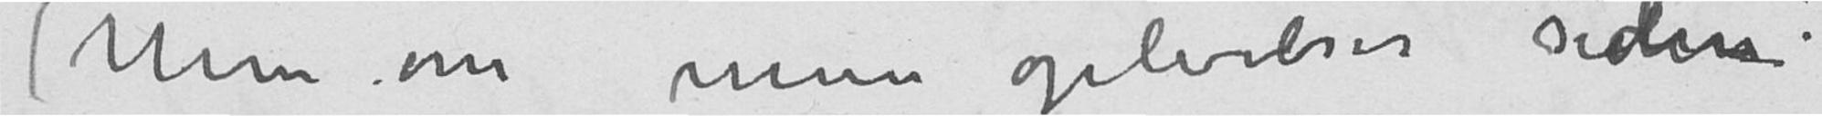(Men om mine oplevelser siden!
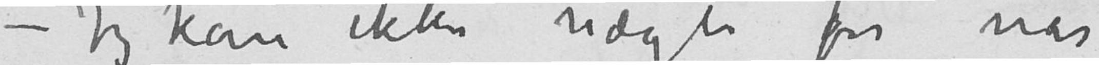Jeg kan ikke nægte for når
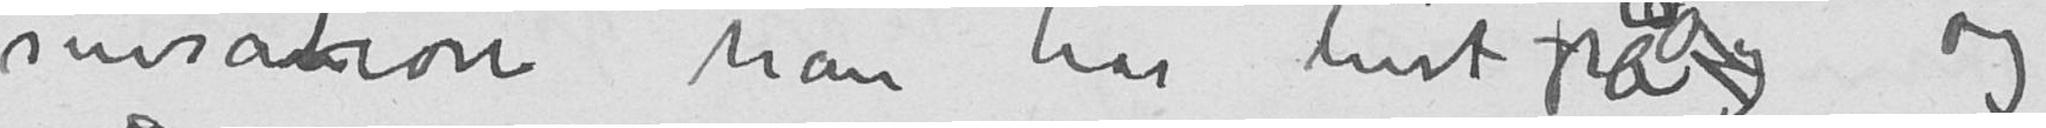sensation han har lurt på og
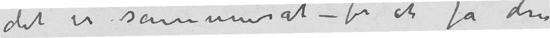det er sammensat – for at få den
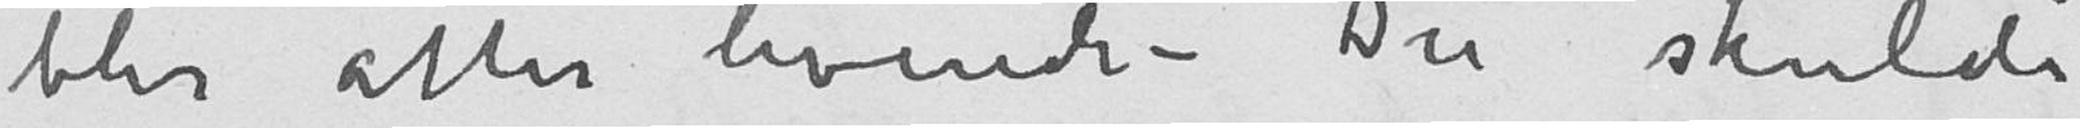blir atter levende – Du skulde
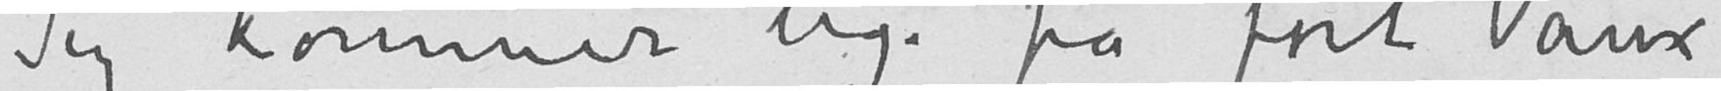Jeg kommer lige fra fort Vaux
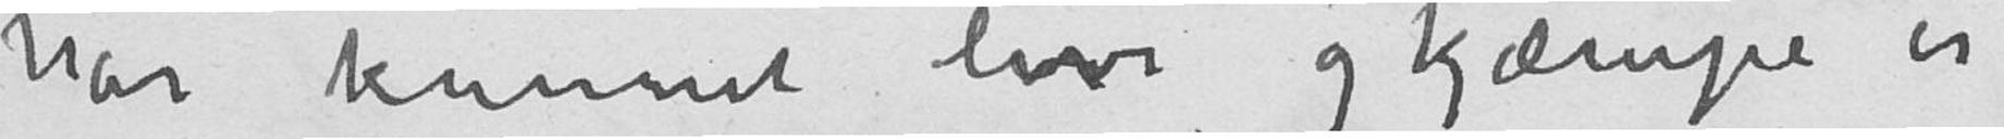har kunnet leve og kjæmpe er
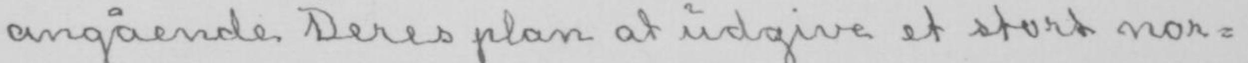angående Deres plan at udgive et stort nor-
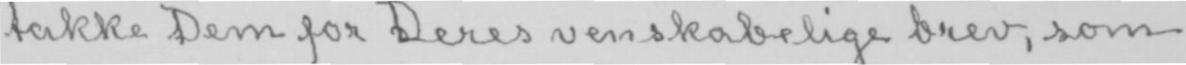takke Dem for Deres venskabelige brev, som
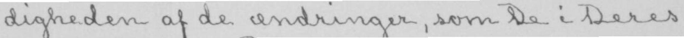digheden af de ændringer, som De i Deres
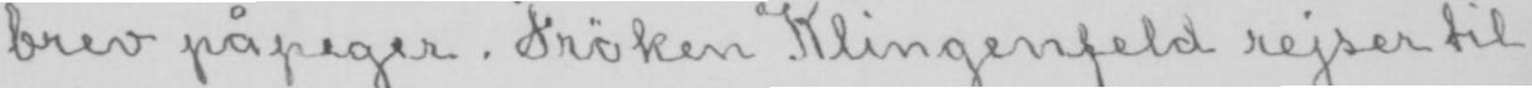brev påpeger. Frøken Klingenfeld rejser til
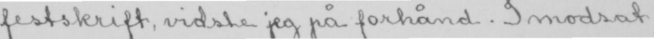festskrift, vidste jeg på forhånd. I modsat
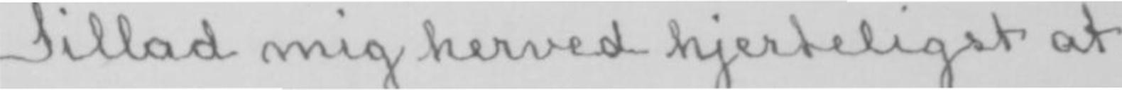Tillad mig herved hjerteligst at
